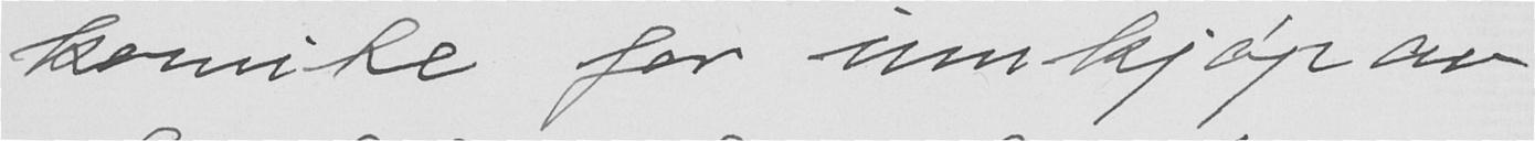komite for innkjøp av
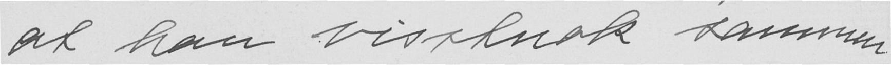at han visstnok sammen
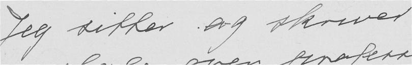Jeg sitter og skriver
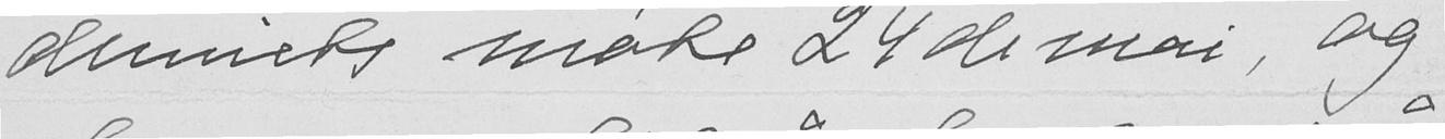demiets møte 24de mai, og
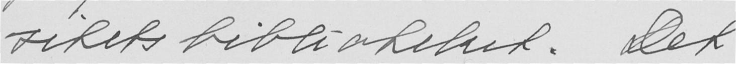sitets biblioteket. Det
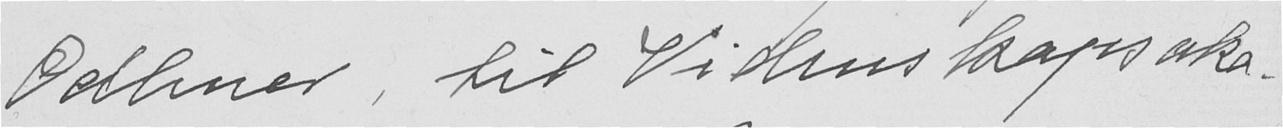Odhner til Videnskapsaka-
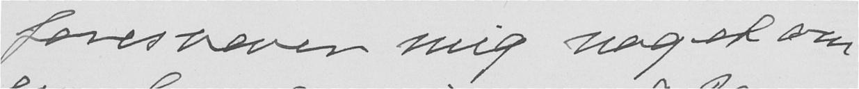foresvæver mig noget om
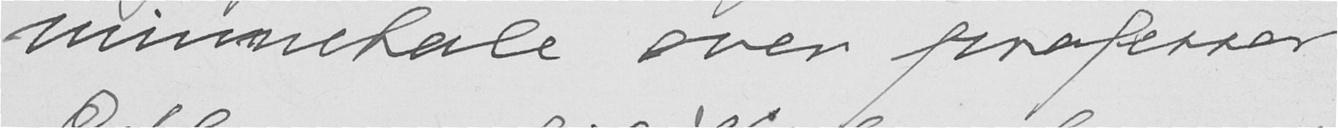minnetale over professor
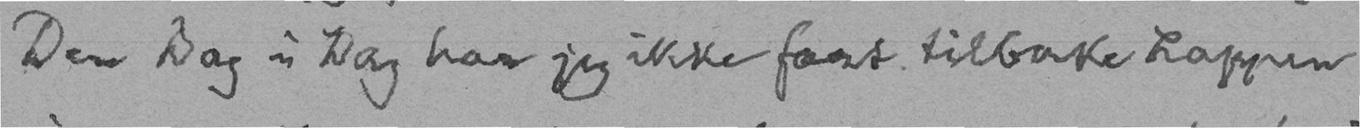Den Dag i Dag har jeg ikke faat tilbake Lappen
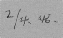2/4. 46.
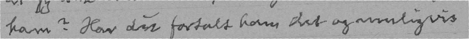ham? Har du fortalt ham det og muligvis
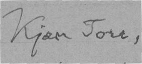Kjære Tore,
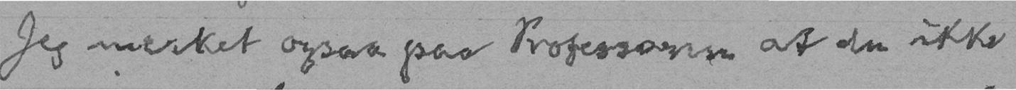Jeg merket ogsaa paa Professoren at du ikke
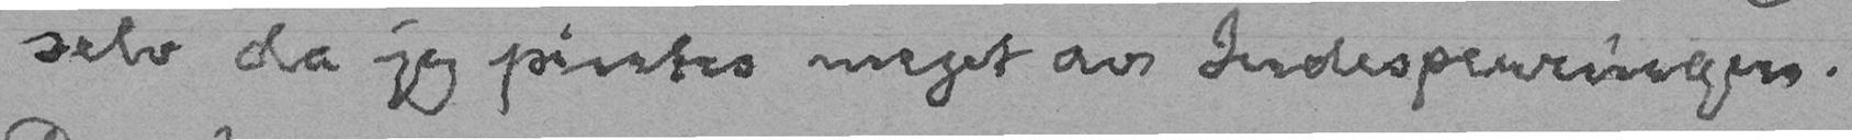selv da jeg pintes meget av Indesperringen.
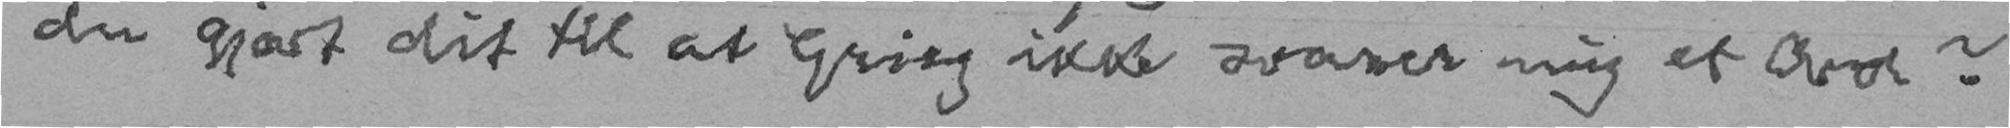du gjort dit til at Grieg ikke svarer mig et Ord?
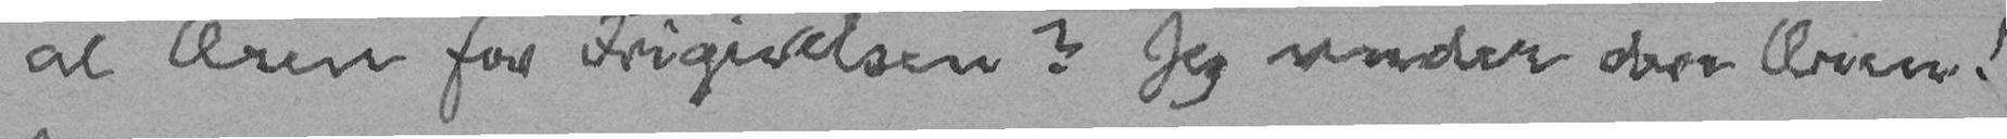al Æren for Frigivelsen? Jeg under dere Æren!
